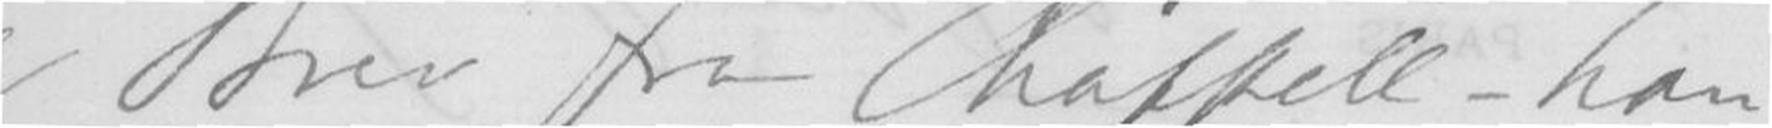jeg Brev fra Chappell - han
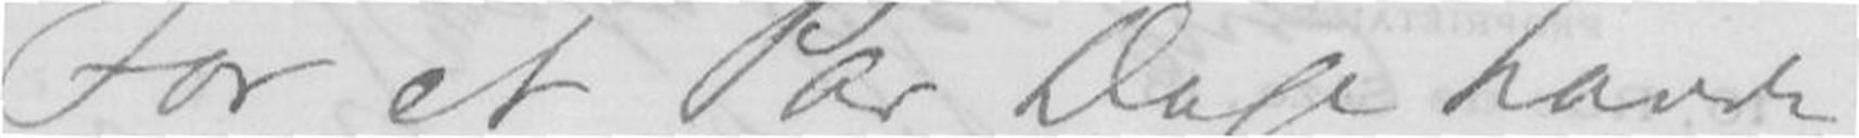For et Par Dage havde
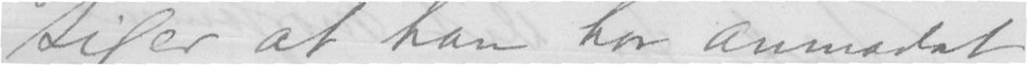siger at han har anmodet
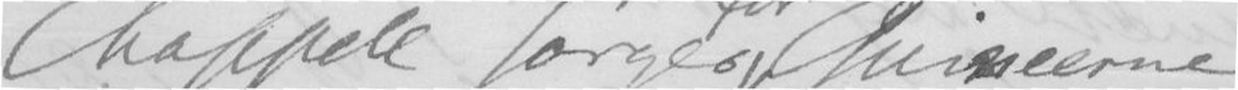Chappell sørger Guineerne og
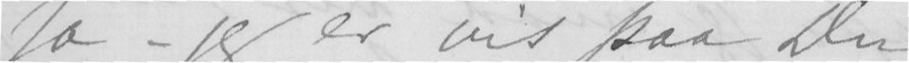Ja - jeg er vis paa Du
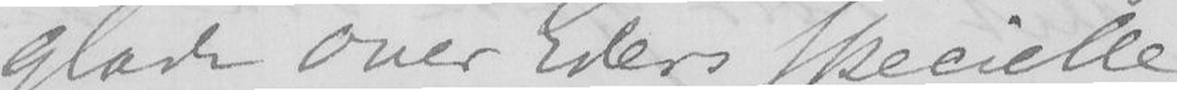glade over Eders specielle
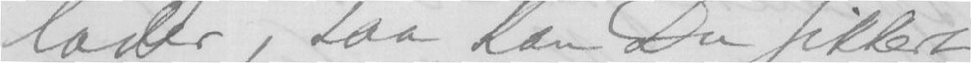lader, saa kan Du sikkert
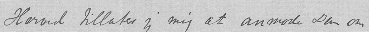Herved tillater jeg mig at anmode Dem om
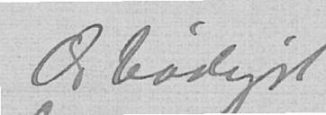Ærbødigst
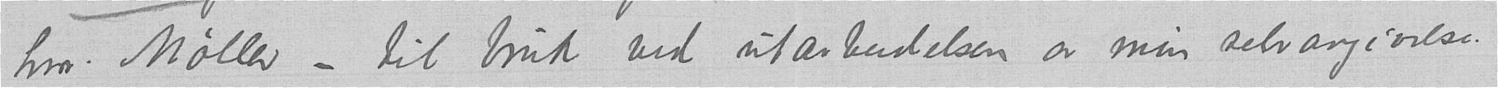herr Møller — til bruk ved utarbeidelsen av min selvangivelse.
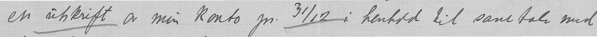en utskrift av min konto pr. 31/12 i henhold til samtale med
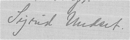Sigrid Undset.
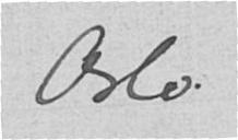Oslo.
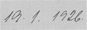19.1.1926.
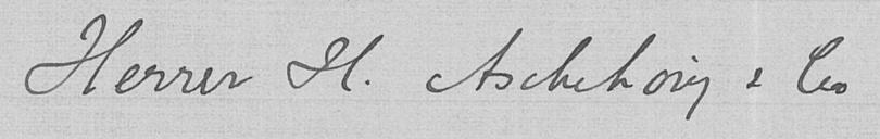Herrer H. Aschehoug & Co.
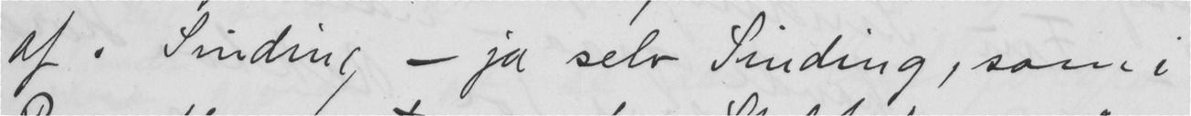af. Sinding - ja selv Sinding, som i
